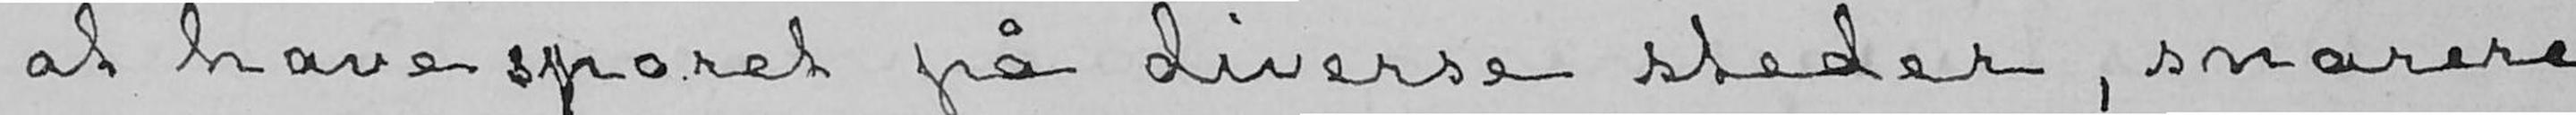at have sporet på diverse steder, snarere
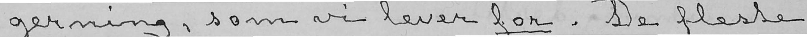gerning, som vi lever for. De fleste
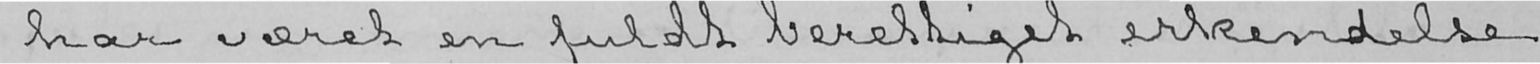har været en fuldt berettiget erkendelse
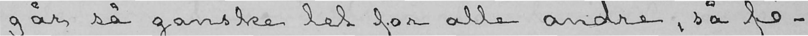går så ganske let for alle andre, så fø-
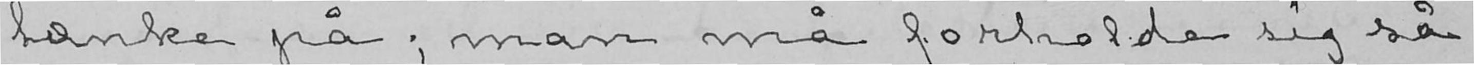tænke på; man må forholde sig så
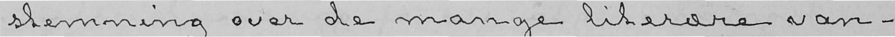stemning over de mange literære van-
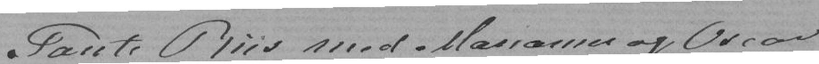Tante Riis med Marianne og Oscar,
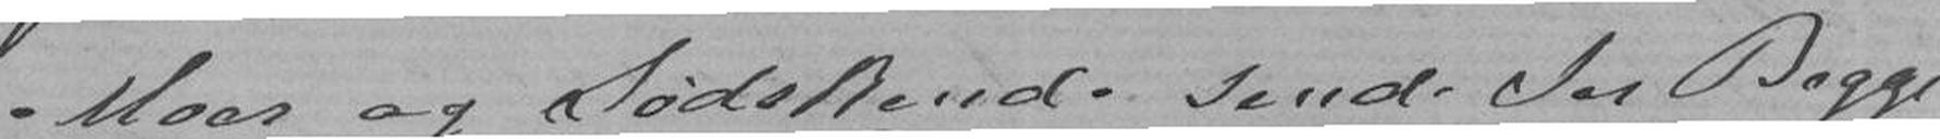Moer og Sødskende sende Jer Begge
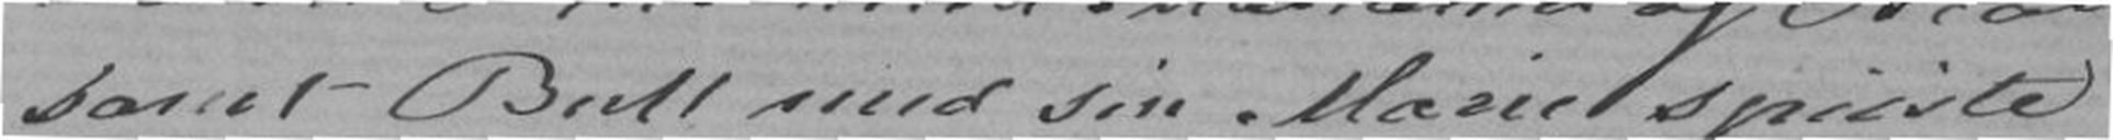samt Bull med sin Marie spiste
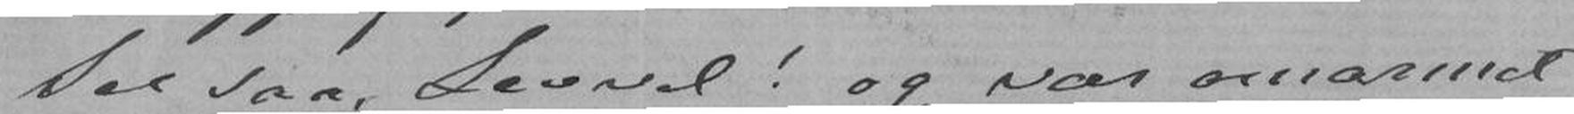See saa, Levvel! og vær omarmet
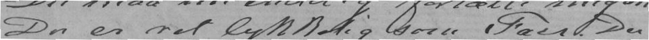Du er ret lykkelig som Faer. Du
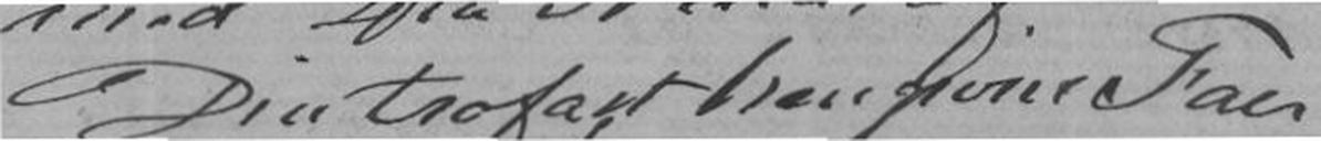Din trofaste hengivne Faer
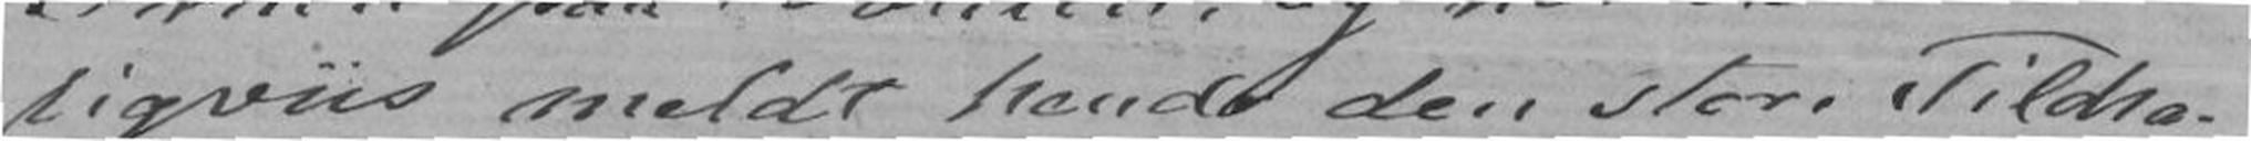ligviis meldt hende den store Tildra-
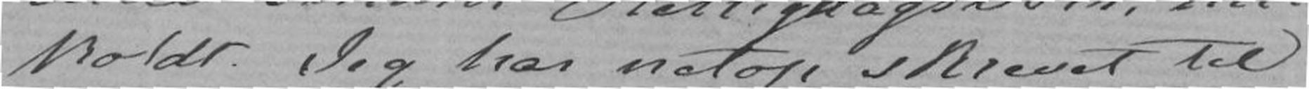koldt. Jeg har netop skrevet til
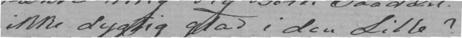ikke dygtig glad i den Lille?
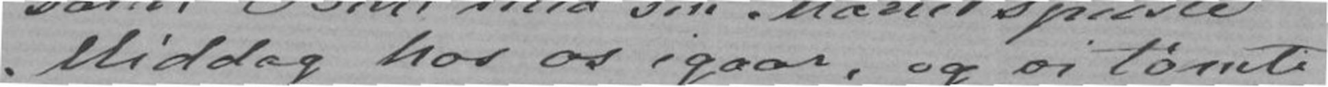Middag hos os igaar, og vi tømte
